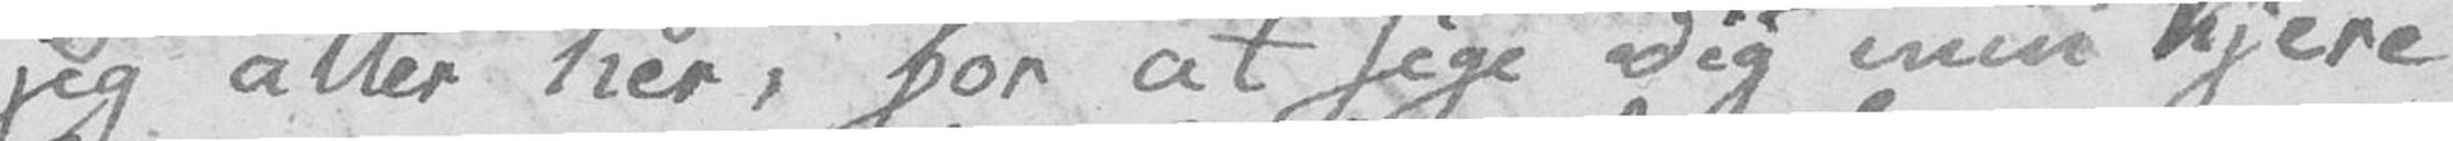jeg atter her, for at sige Dig min kjere
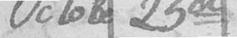Octobe 25d
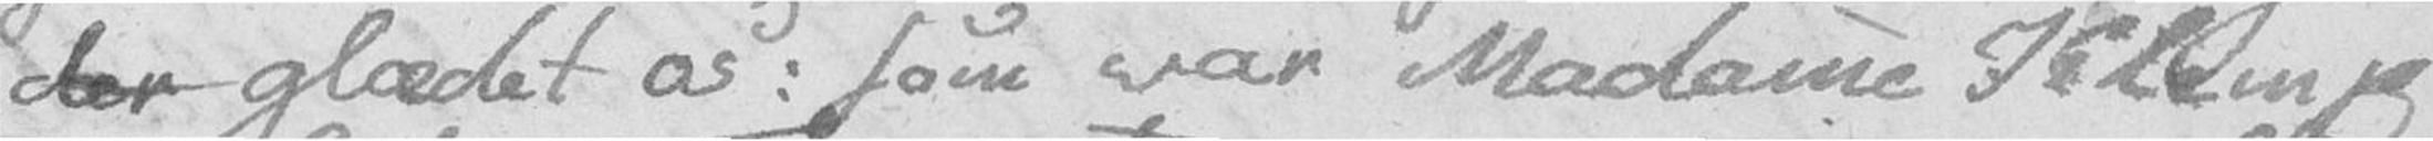der glædet os: som var Madamme Klemp
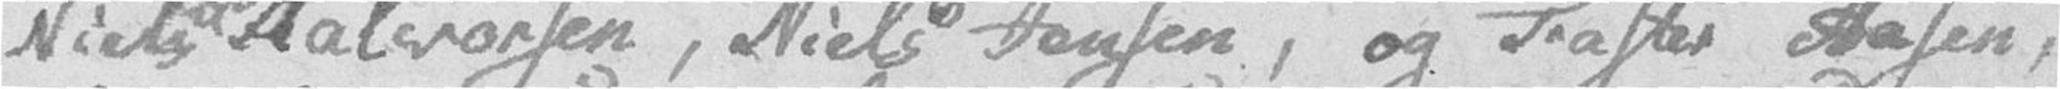Niels Halvorsen, Niels Jansen, og Faster Aasen,
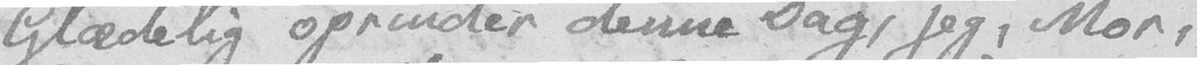Glædelig oprinder denne Dag, jeg, Mor,
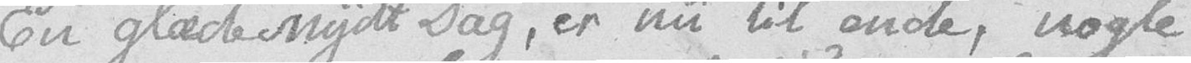En glæde nydt Dag, er nu til ende, nogle
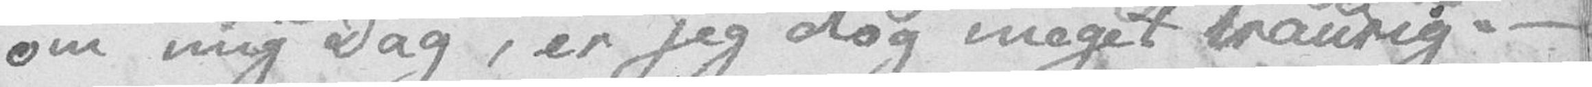om mig Dag, er jeg dog meget traurig. –
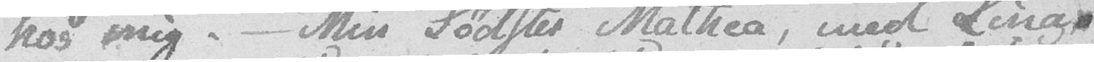hos mig. – Min Sødster Mathea, med Lina,
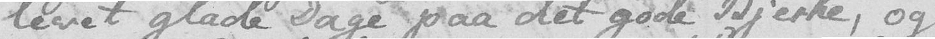levet glade Dage paa det gode Bjerke, og
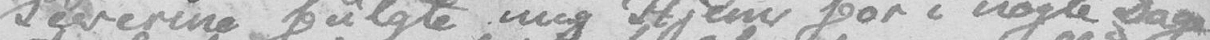Severine fulgte mig Hjem for i nogle Dage
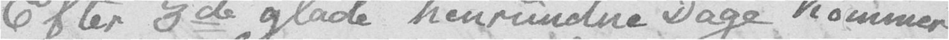Efter 3de glade henrundne Dage kommer
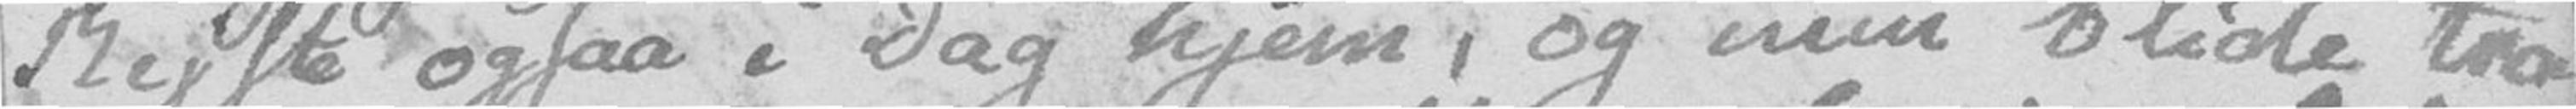Rejste ogsaa i Dag hjem, og min blide tro
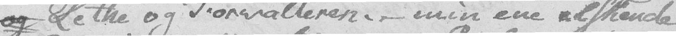og Lethe og Forvalteren. – min ene elskende
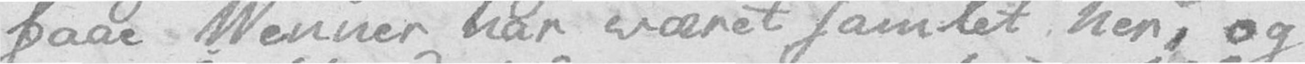faae Venner har været samlet her, og
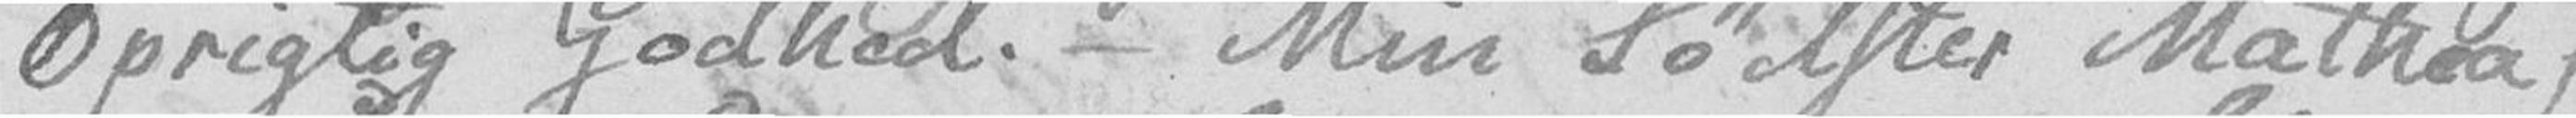Oprigtig Godhed. – Min Sødster Mathea,
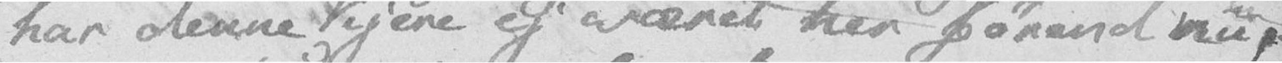har denne kjere ej været her førend nu.
